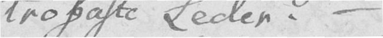trofaste Leder. –
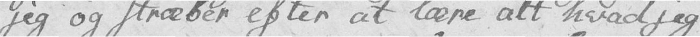jeg og stræber efter at lære alt hvad jeg
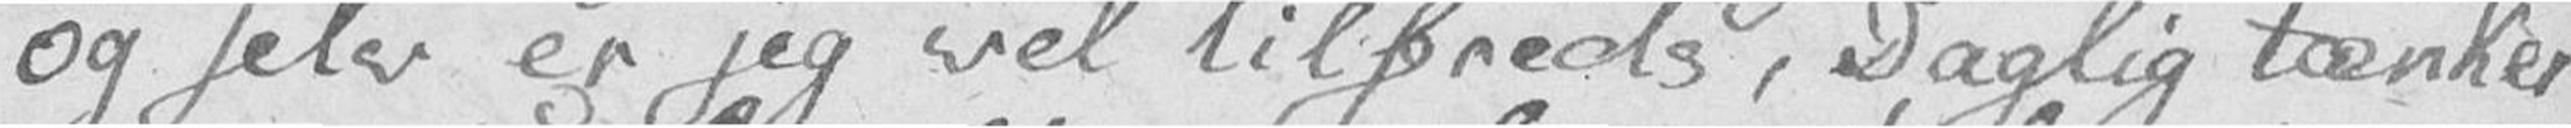og selv er jeg vel tilfreds, Daglig tænker
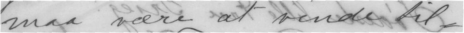maa være at vende til-
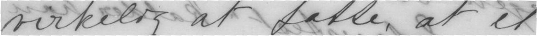virkelig at fatte, at et
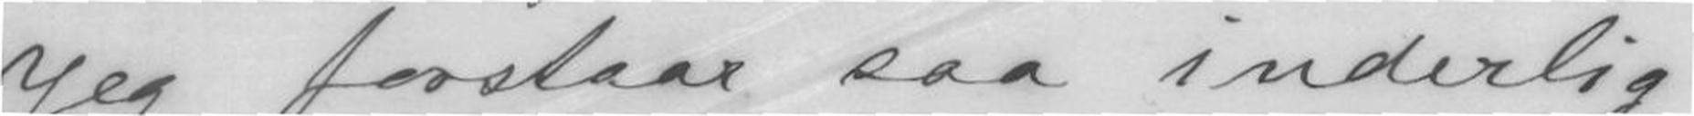Jeg forstaar saa inderlig
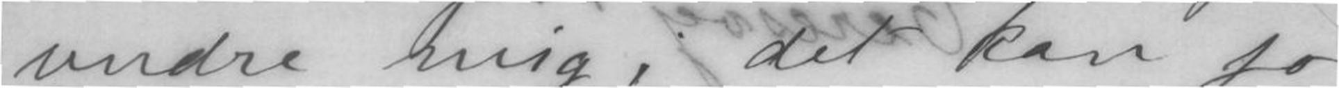undre mig, det kan jo
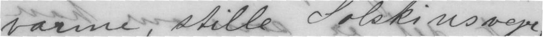varme, stille Solskinsvejr,
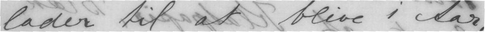lader til at blive i Aar,
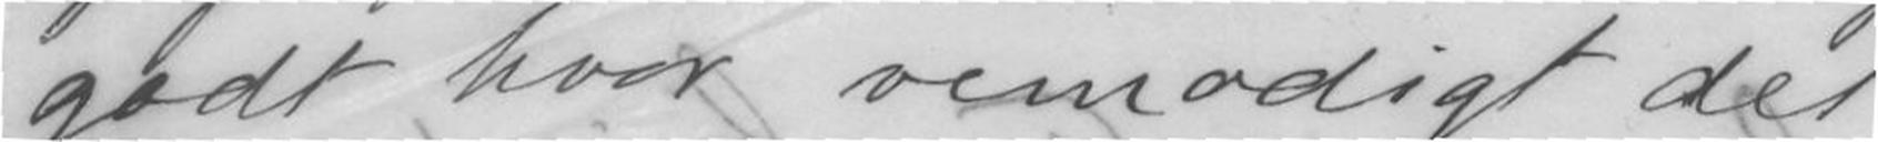godt hvor vemodigt det
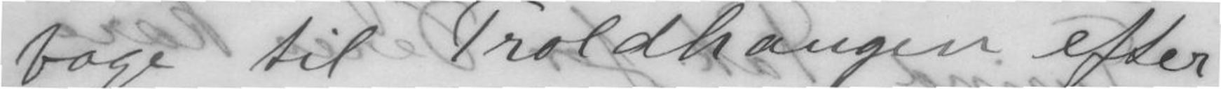bage til Troldhaugen efter
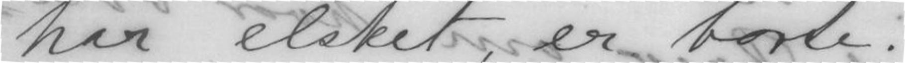har elsket, er borte.
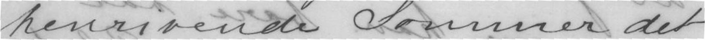henrivende Sommer det
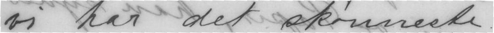vi har det skønneste
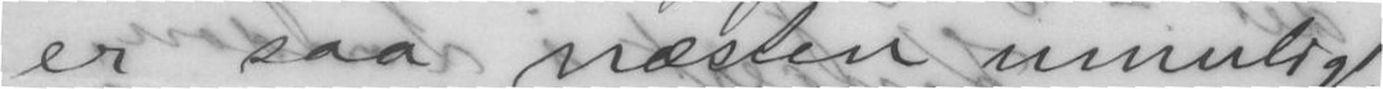er saa næsten umuligt
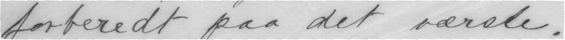forberedt paa det værste.
